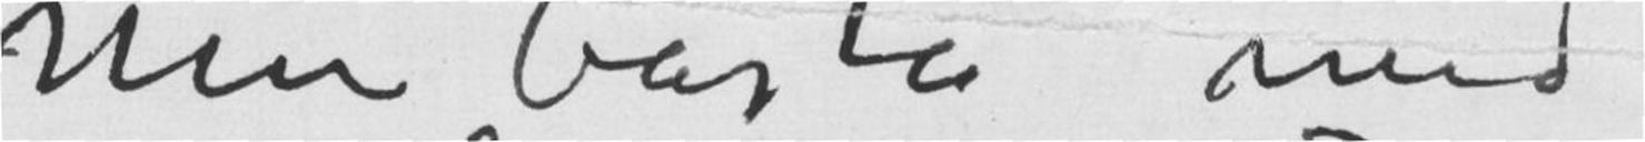Men basta med
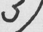3)
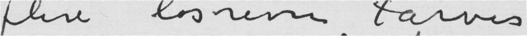flere løsrevne Farver
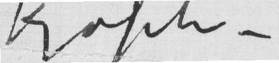kjøpte –
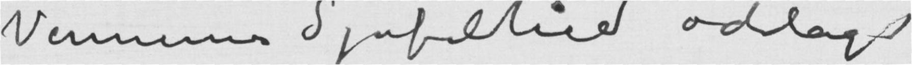Vennenes Sjofelthed ødelagt
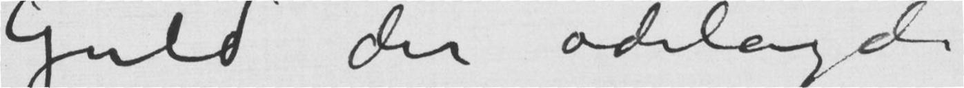Guld der ødelagde
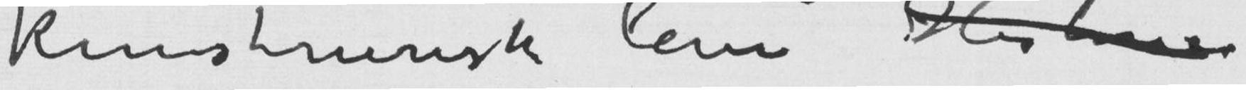kunstnerisk leve Historien
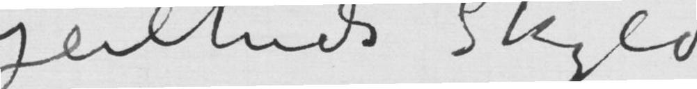Geilheds Skyld
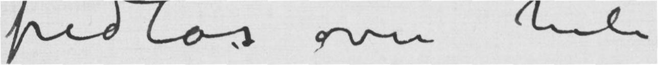fredløs over hele
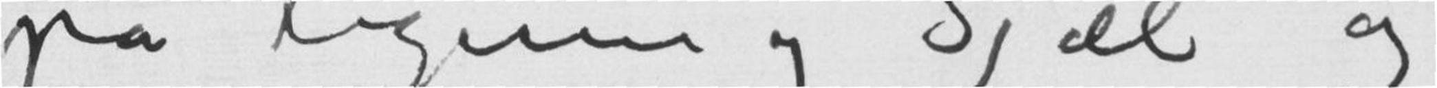på Legeme og Sjæl og
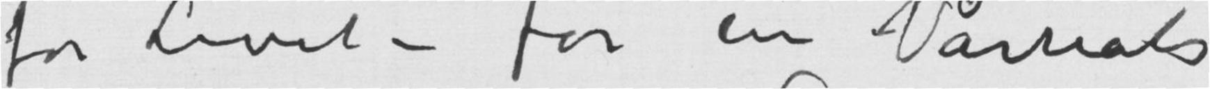for Livet – for en Vårnats
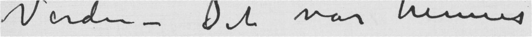Verden – Det var hennes
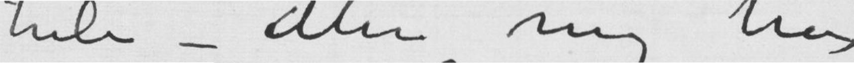hele – Men mig har
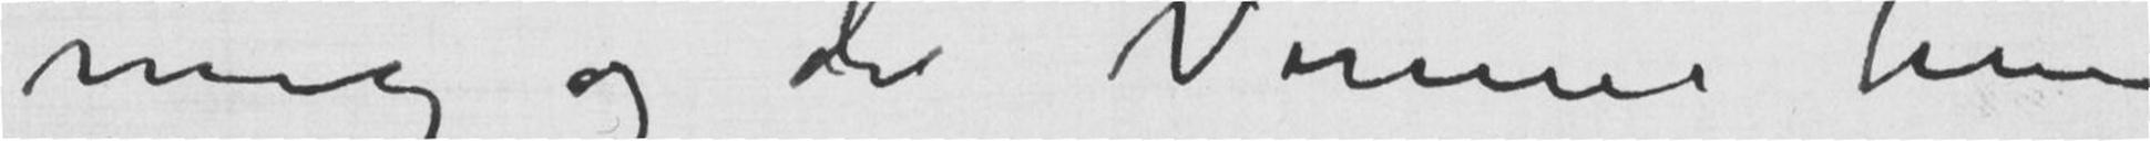mig og de Venner hun
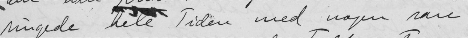ringede hele Tiden med nogen rare
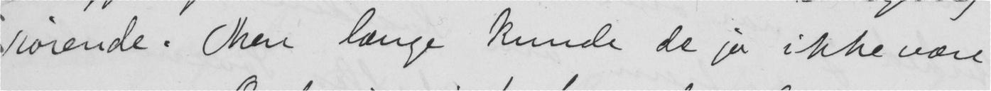rørende. Men længe kunde de jo ikke være
